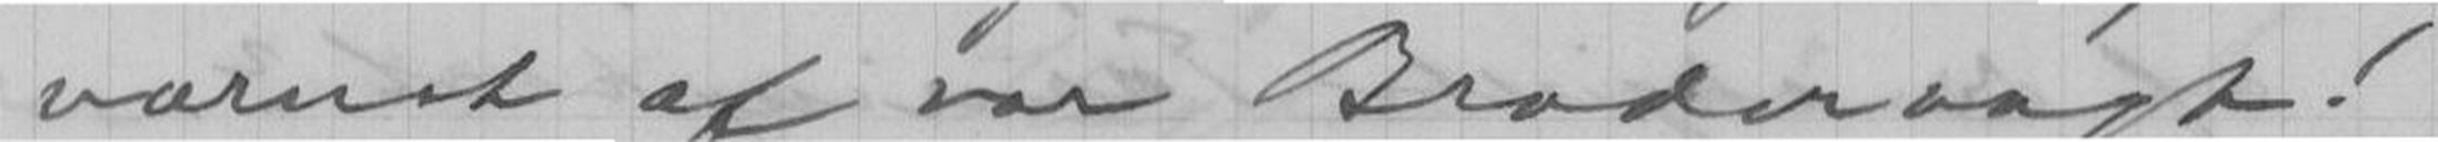varmt af vor Brodervagt!
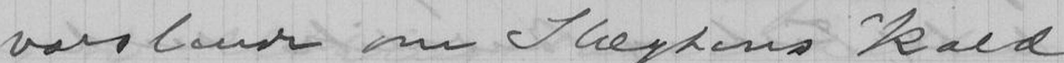varslende om Slægtens kald
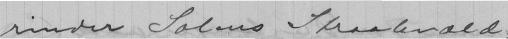rinder Solens Straalevæld;
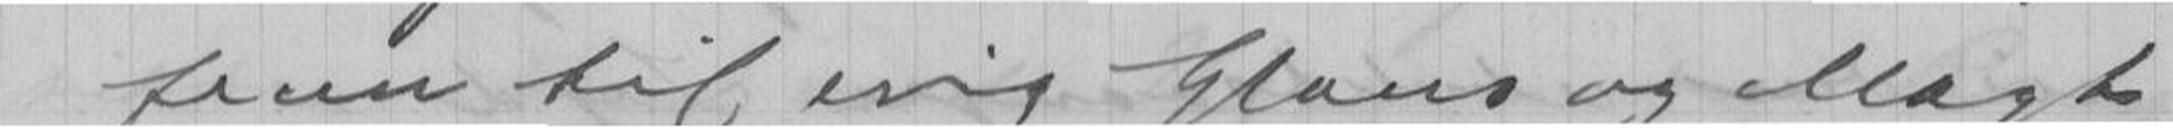frem til evig Glans og Magt
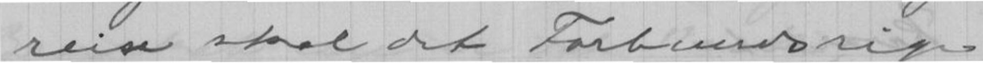reise skal det Forbundsrige,
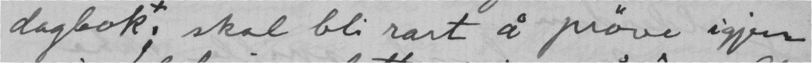dagbok; skal bli rart å prøve igjen
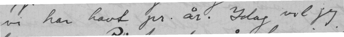vi har havt pr. år. Idag vil jeg
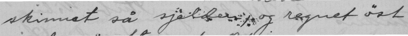skinnet så sjelden, og regnet øst
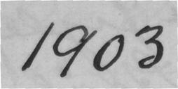1903
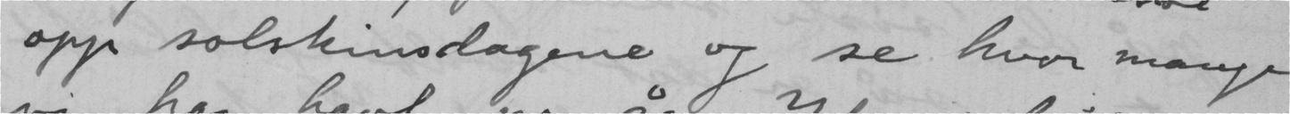opp solskinsdagene og se hvor mange
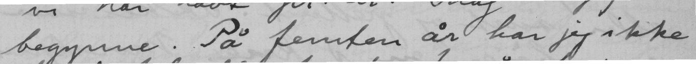begynne. På femten år har jeg ikke
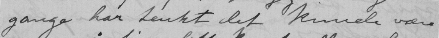gange har tenkt det kunde være
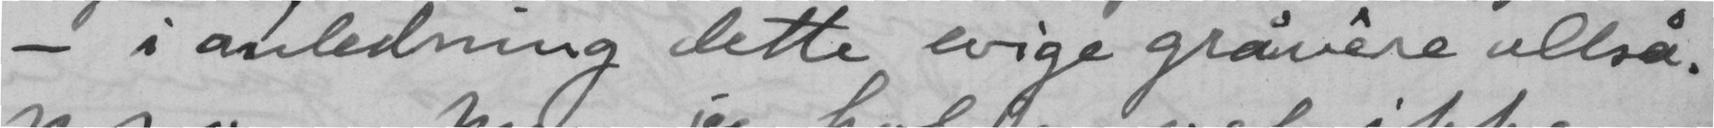- i anledning dette evige gråvêre altså.
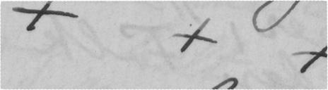x x x
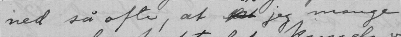ned så ofte, at  jeg mange
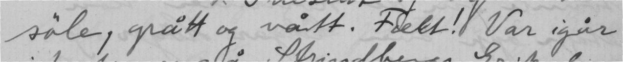søle, grått og vått. Fælt! Var igår
